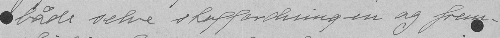både selve skaffordningen og frem-
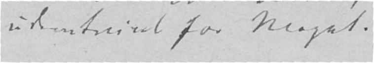udentvivl for Meget.
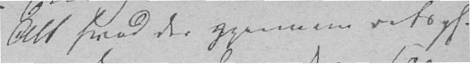Alt hvad der gjennem retsph.
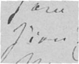sion
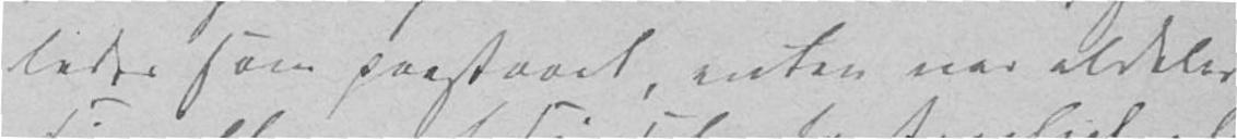ledes som paastaaet, enten var aldeles
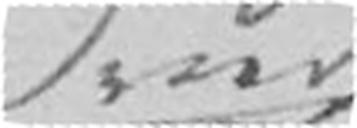ved
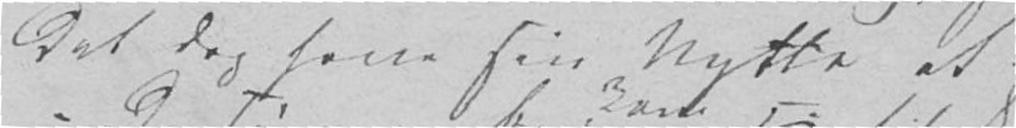det dog have sin Nytte at
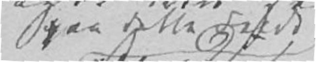paa dette Feldt
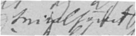tvivlsomt
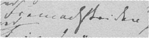Fremadskriden
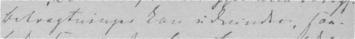Betragtninger kan udvindes, saa-
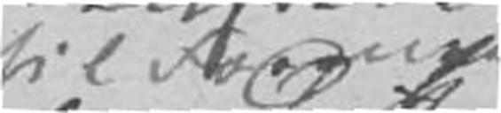til Formaal
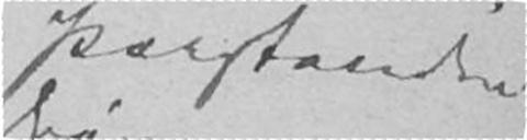Paastanden
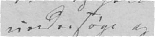undersøge og
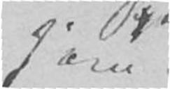som
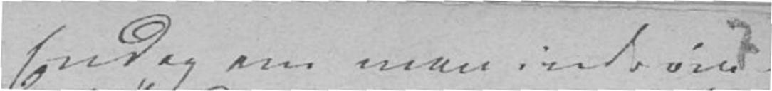Endog om man indrøm-
